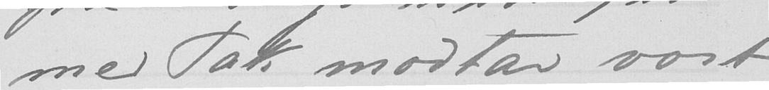med Tak modtar vort
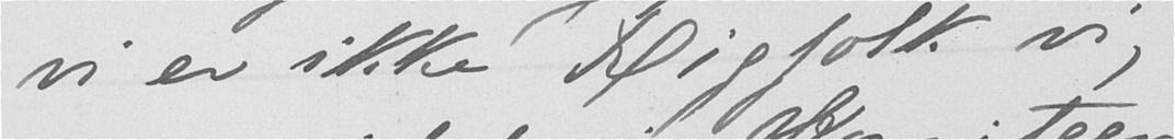vi er ikke Rigfolk vi,
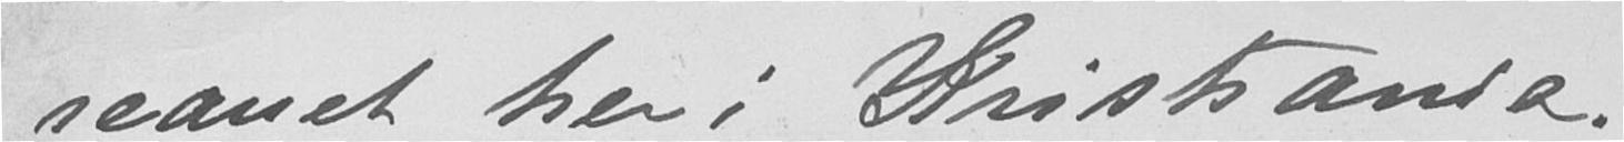reauet her i Kristiania.
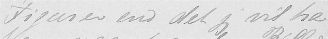Figurer end det jeg vil ha
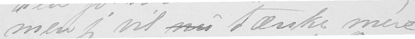men jeg vil nu tænke mere
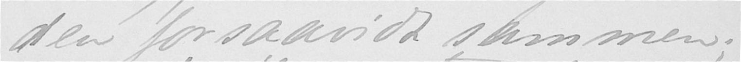den forsaavidt sammen;
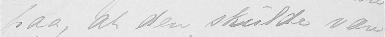paa, at den skulde være
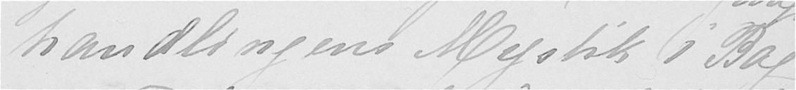handlingens Mystik i Bag-
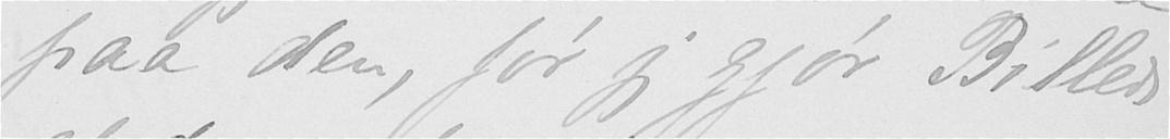paa den, før jeg gjør Billede
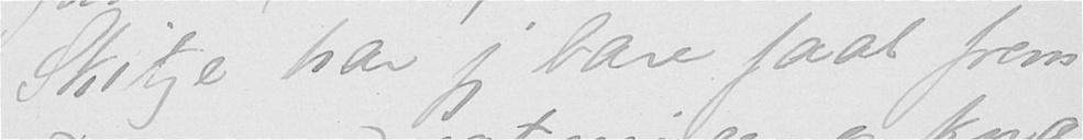Skitze har jeg bare faat frem
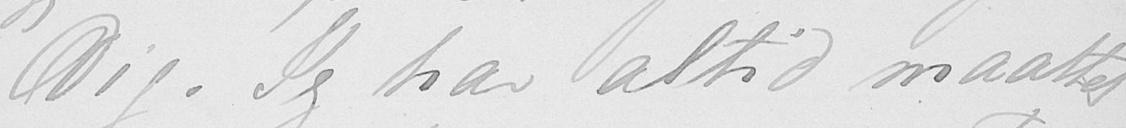Dig. Jeg har altid maatte
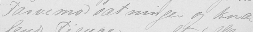Farvemodsætninger og knæ-
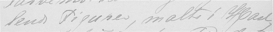lende Figurer, malte i Hast,
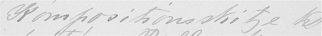Kompositionskitze til
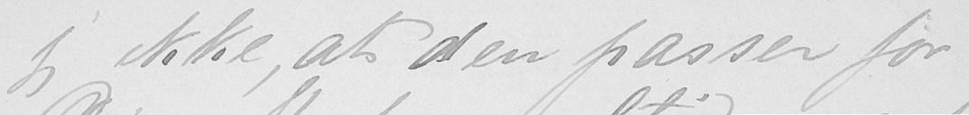jeg ikke, at den passer for
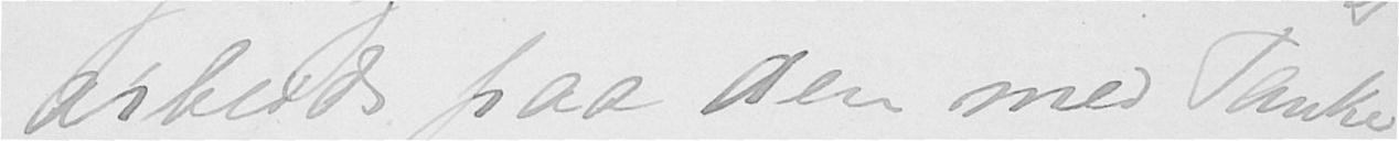arbeide paa den med Tanke
